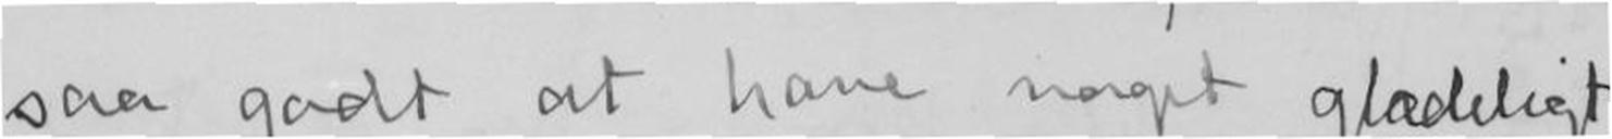saa godt at have noget glædeligt
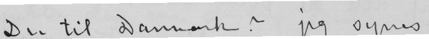Du til Danmark? jeg synes
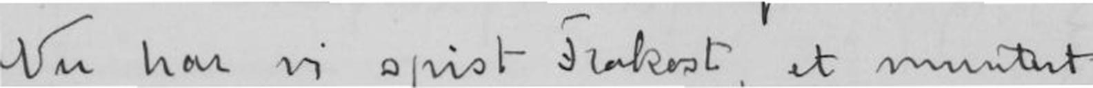Nu har vi spist Frokost, et muntert
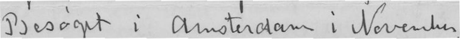Besøget i Amsterdam i November,
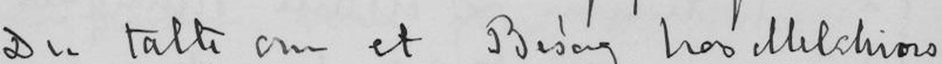Du talte om et Besøg hos Melchios
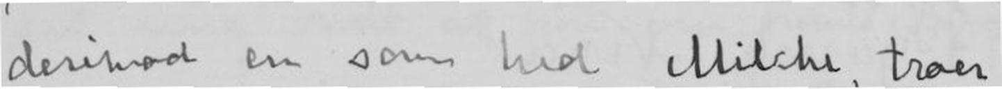derimod en som hed Milche, troer
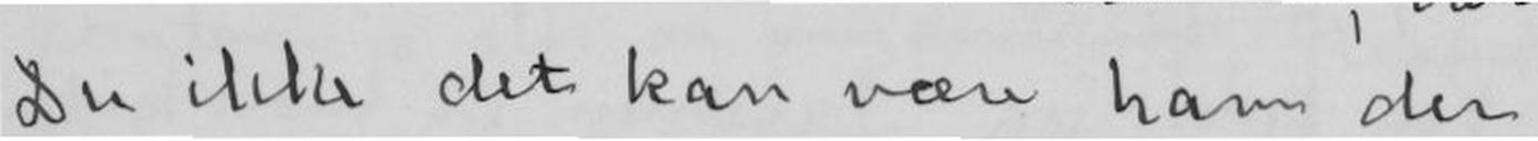Du ikke det kan være ham der
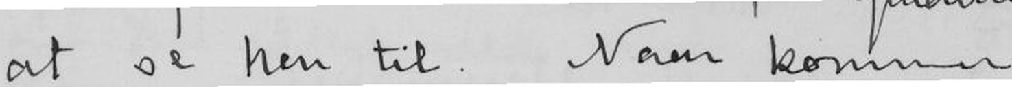at se hen til. Naar kommer
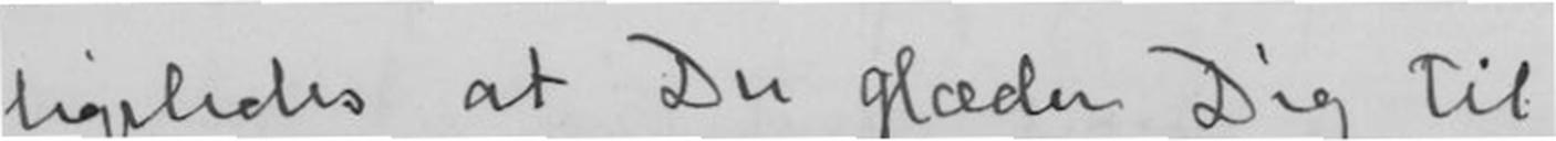ligeledes at Du glæder Dig til
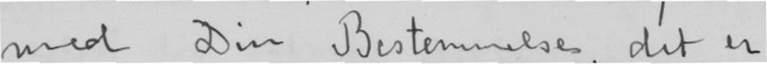med Din Bestemmelse, det er
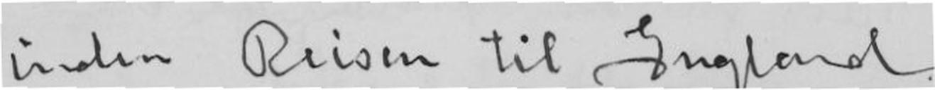unden Reisen til England.
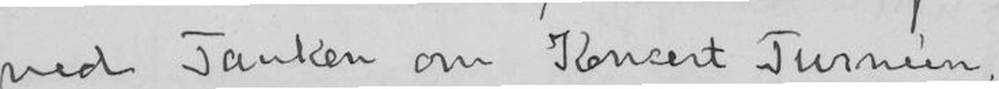ved Tanken om Koncert Turneen,
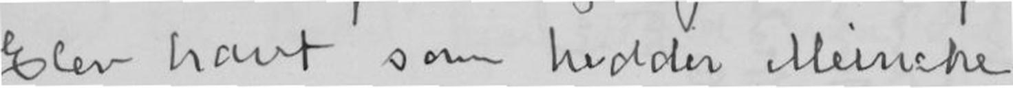Elev havdt som hedder Meinche,
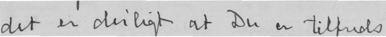det er deiligt at Du er tilfreds
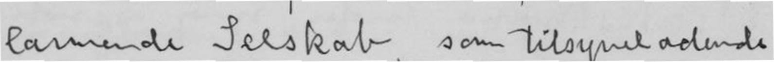larmende Selskab, som tilsyned ladende
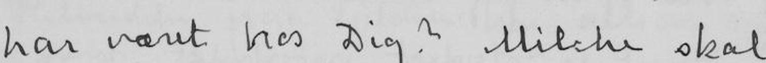har været hos Dig? Milche skal
